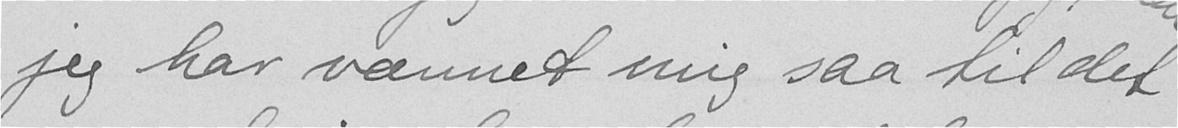jeg har vænnet mig saa til det
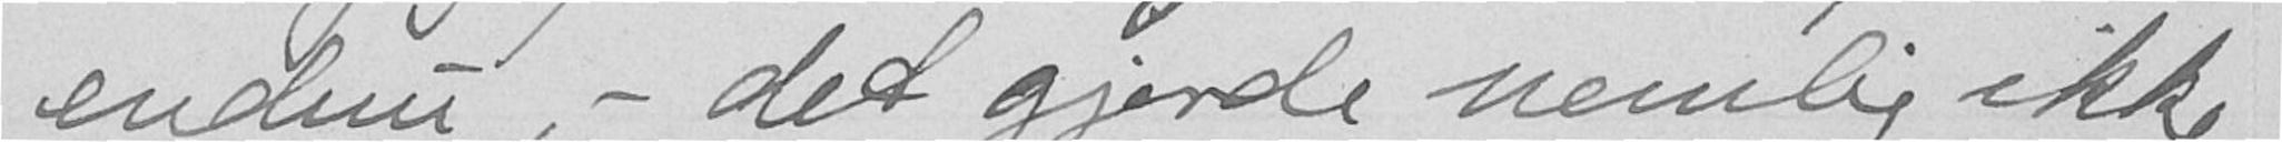endnu, - det gjorde nemlig ikke
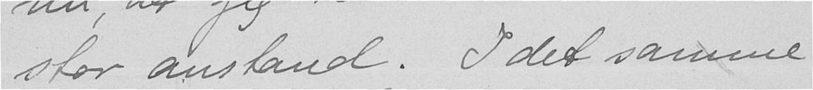stor anstand. I det samme
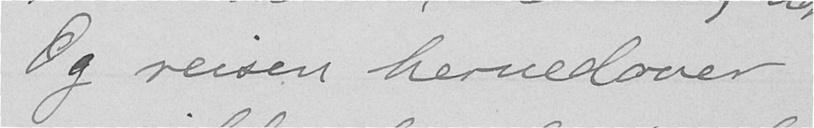Og reisen hernedover
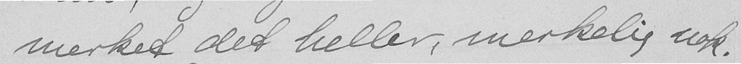merket det heller, merkelig nok.
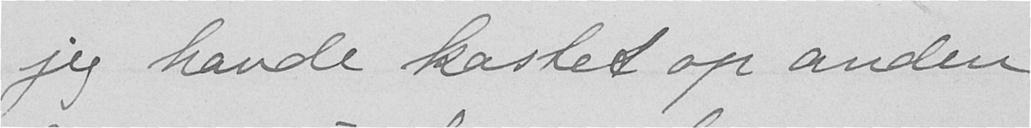jeg havde kastet op anden
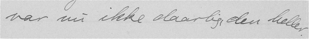var nu ikke daarlig den heller.
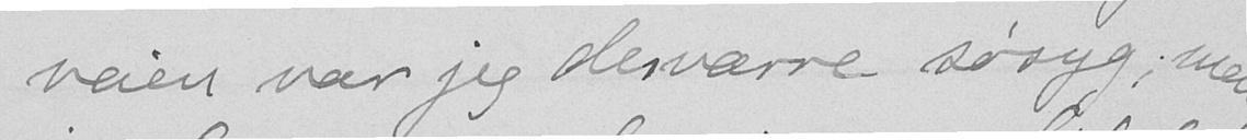veien var jeg desværre søsyg men
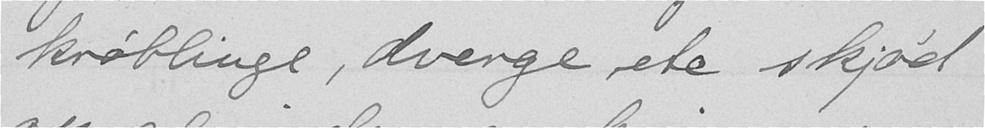krøblinge, dverge etc skjød
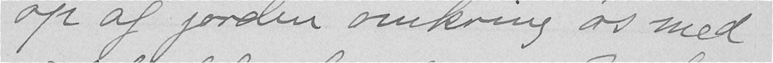op af jorden omkring os med
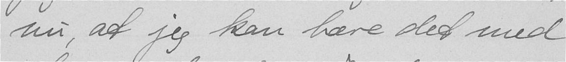nu at jeg kan bære det med
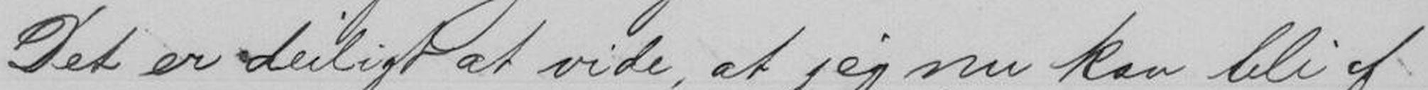Det er deiligt at vide, at jeg nu kan bli af
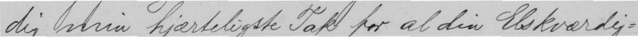dig min hjærteligste Tak for al din Elskværdig-
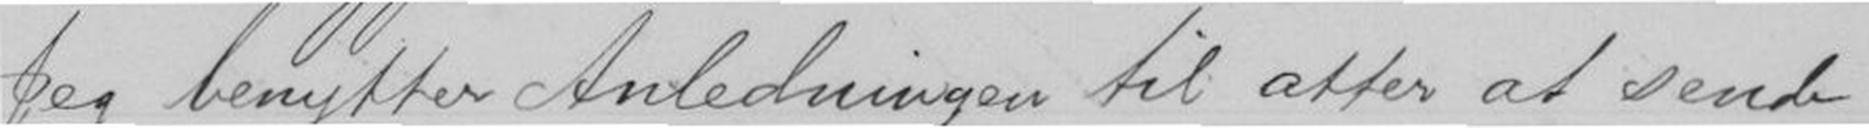Jeg benytter Anledningen til atter at sende
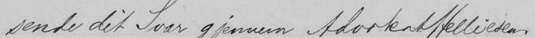sende dit Svar gjennem Advokat Helliesen.
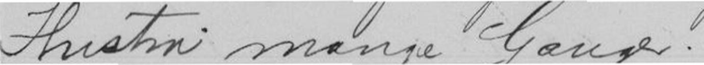Hustru mange Gange.
